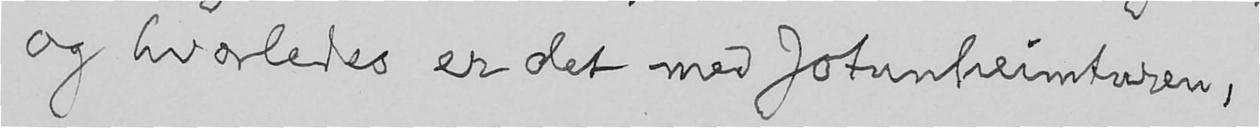og hvorledes er det med Jotunheimturen,
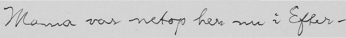Mama var netop her nu i Efter-
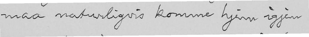maa naturligvis komme hjem igjen
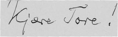Kjære Tore!
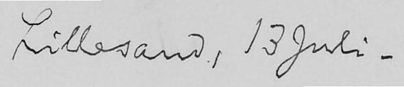Lillesand, 13 Juli.
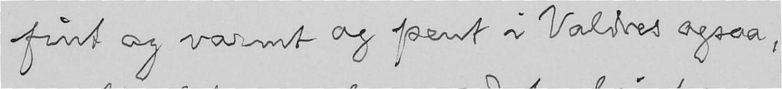fint og varmt og pent i Valdres ogsaa,
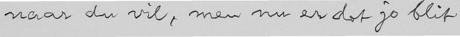naar du vil, men nu er det jo blit
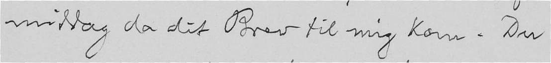middag da dit Brev til mig kom. Du
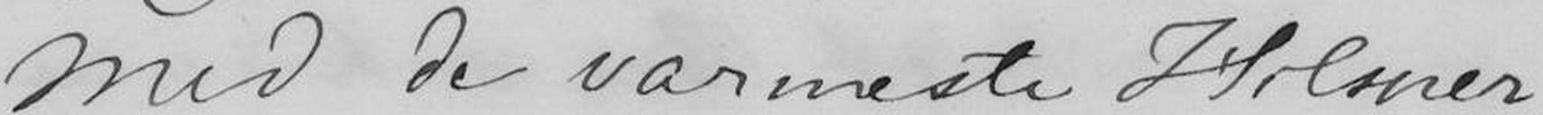Med de varmeste Hilsner
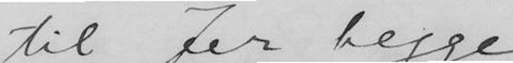til Jer begge
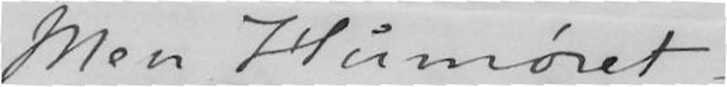Men Humøret -
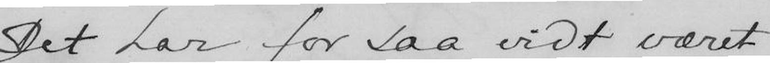Det har for saa vidt været
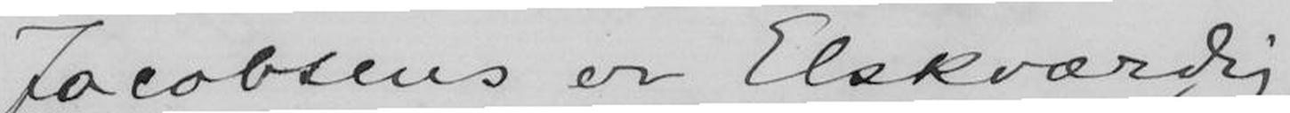Jacobsens er Elskværdig-
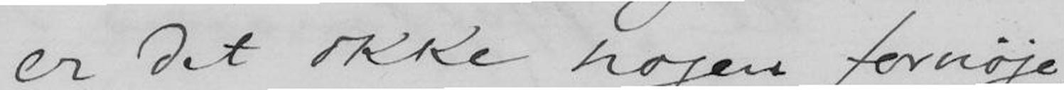er det ikke nogen fornøje-
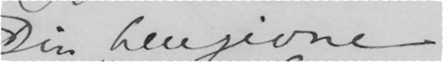Din hengivne
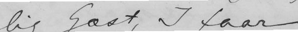lig Gæst, I faar.
